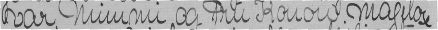var Mimmi og Fru Konow mageløse
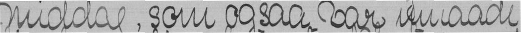middag, som ogsaa var umaade
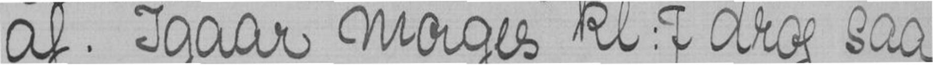af. Igaar morges kl:7 drog saa
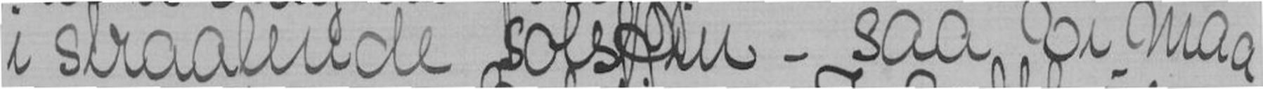i straalende solskin - saa vi maa
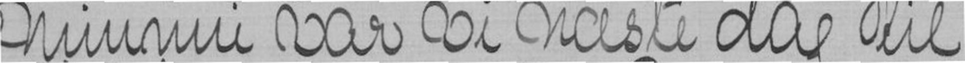Mimmi var vi næste dag til
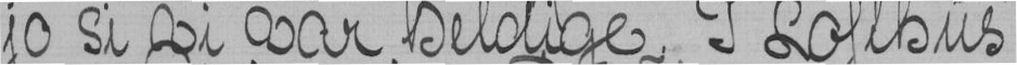jo si vi var heldige I Lofthus
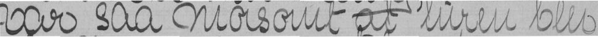var saa morsomt at turen blev
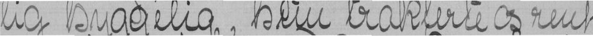lig hyggelig, hun trakterte os rent
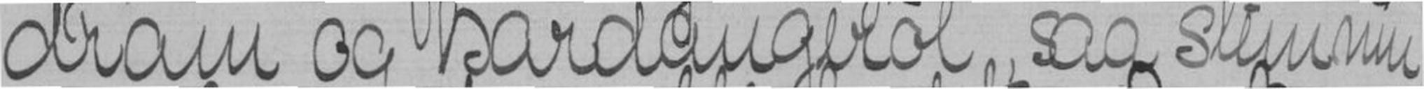dram og hardangerøl, saa stemnin
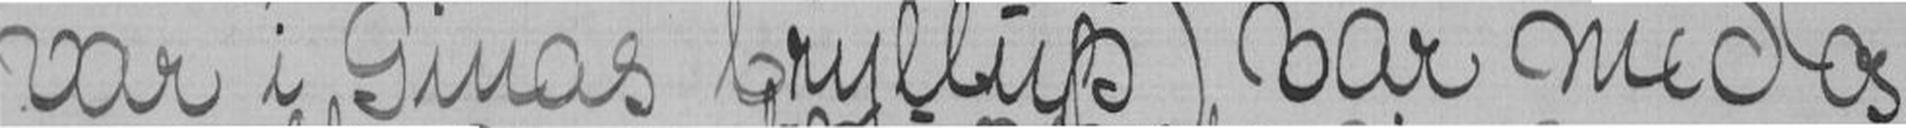var i Ginas bryllup var med os
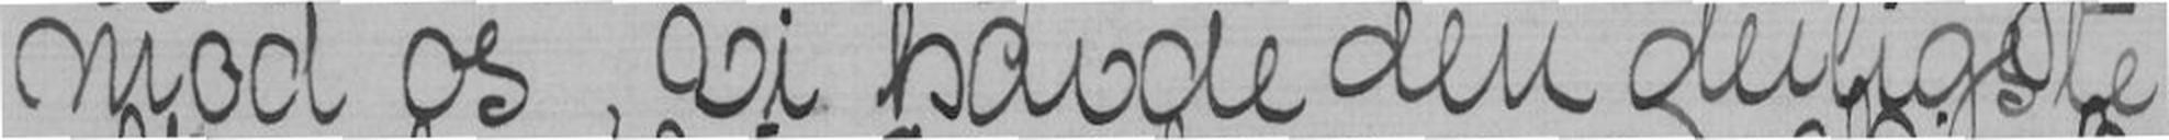mod os, vi havde den deiligste
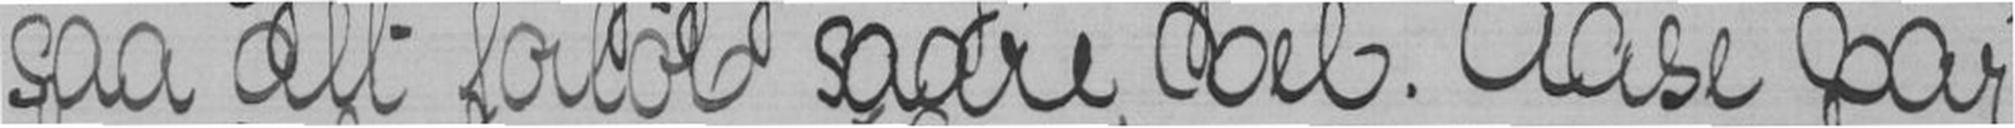saa alt forløb saare vel. Aase var
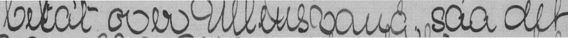betat over Ullensvang, saa det
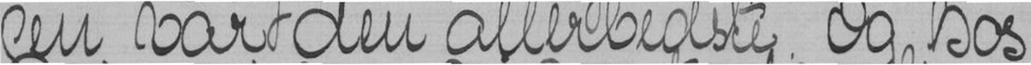gen var den allerbedste. Og hos
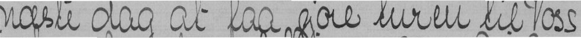næste dag at faa gjøre turen til Voss
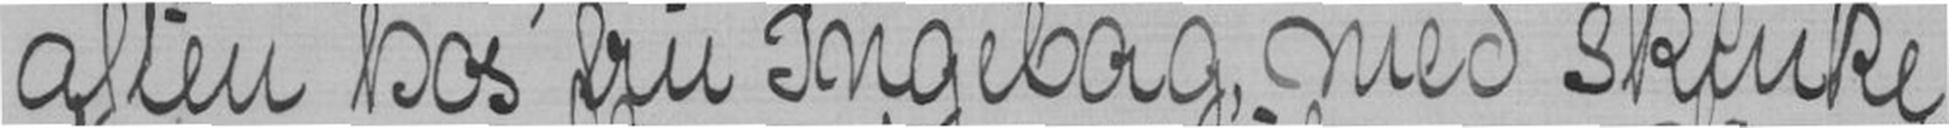aften hos fru Ingeborg, med skinke
